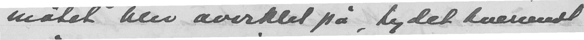møtet blir avviklet på, tydet tvertimot
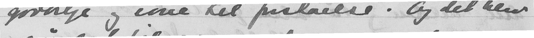godvilje og evne til forståelse. Og det blev
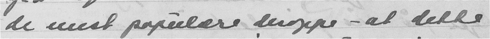de mest populære deroppe - at dette
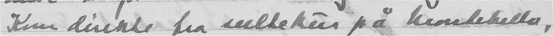Kom direkte fra seiltekus på kiontehello,
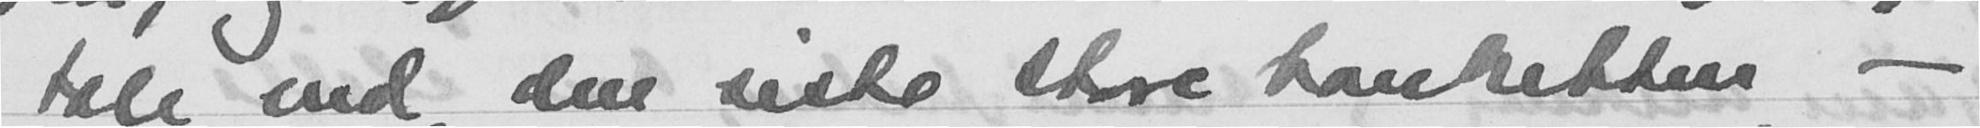tale ved den siste store banketten -
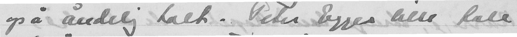også åndelig talt. Peter Egges lille tale
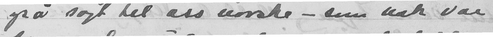også sagt til oss norske - som nok var
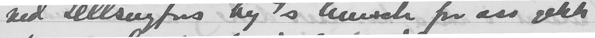ved Hellsingfors by's lmst for oss gikk
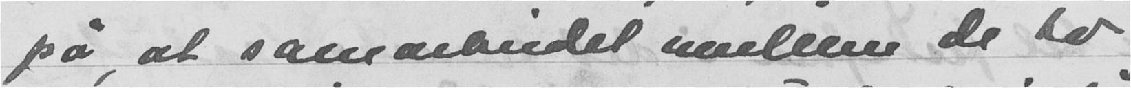på, at samarbeidet mellom de to
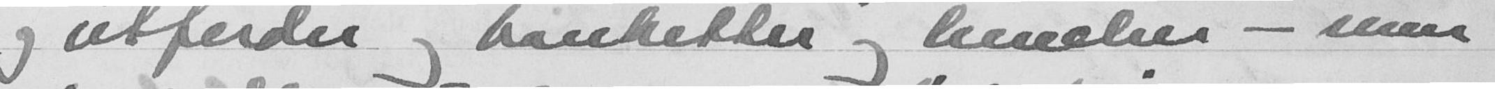og utferder og banketter og lemoleer - men
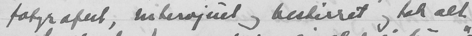fotgrafert, intervjuet og beskisset og tok alt
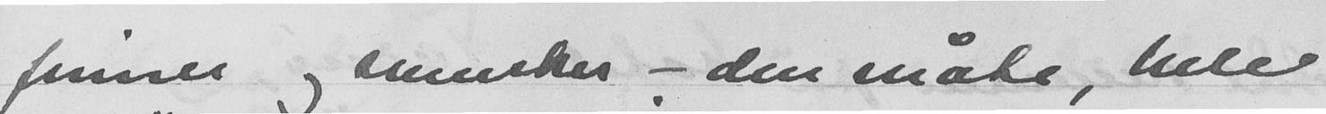finner og svensker - den måte, hele
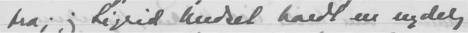bra; og Sigrid Undset holdt en nydelig
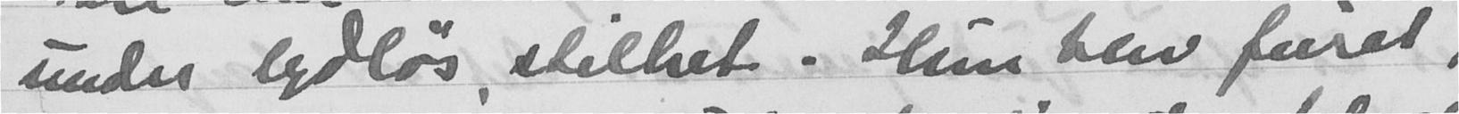under lydløs stillhet. Hun blir furet,
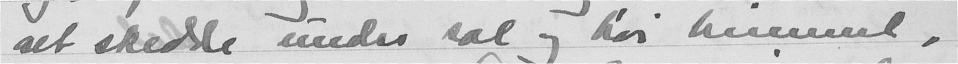det skedde under sol og høi himmel,
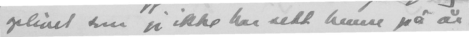oplivet som jeg ikke har sitt henne på år.
